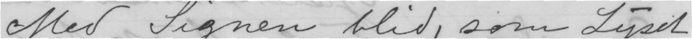Med Signen blid, som Lyset
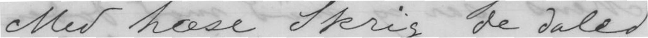Med hæse Skrig de daler
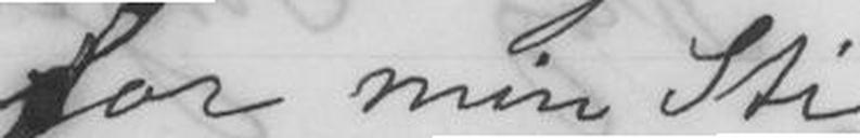for min Sti.
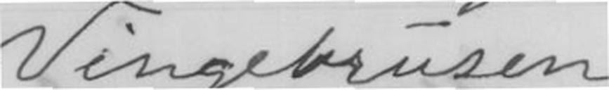Vingebrusen.
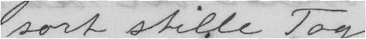sort stille Tog
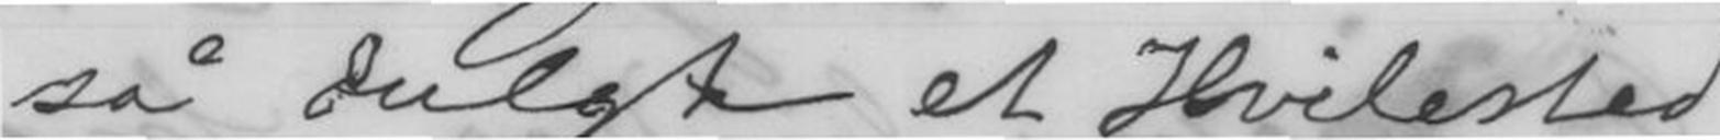så dulgt et Hvilested
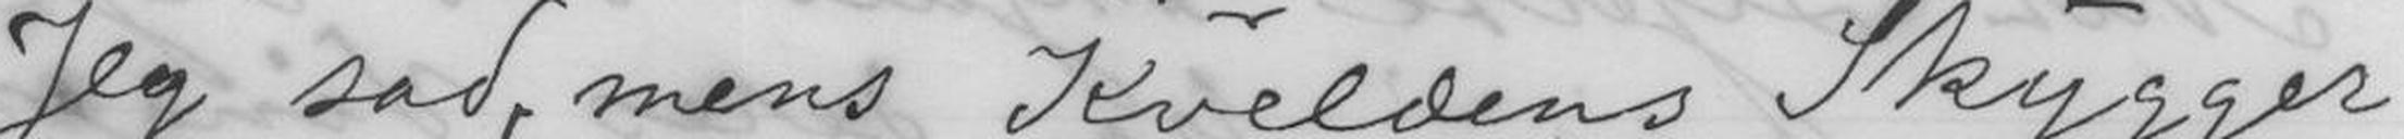Jeg sad, mens Kveldens Skygger
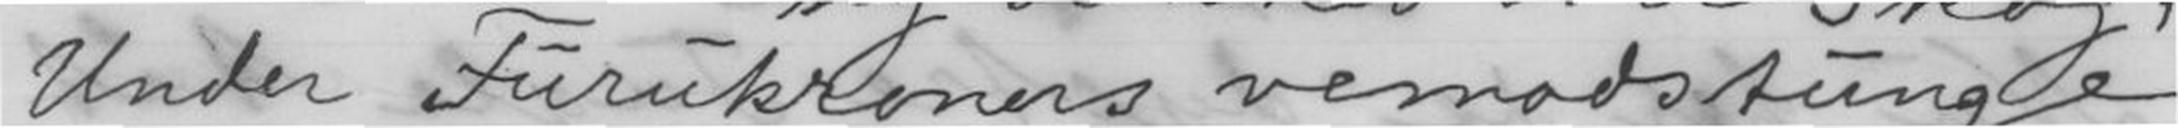Ender Furukroners vemodstunge
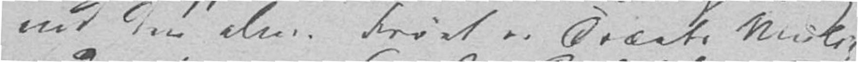end den alm. Frøet er Træets Mulig-
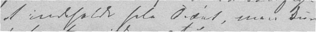at indeholde hele Træet, men kun
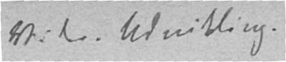Videre Udvikling.
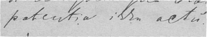potentia ikke actu.
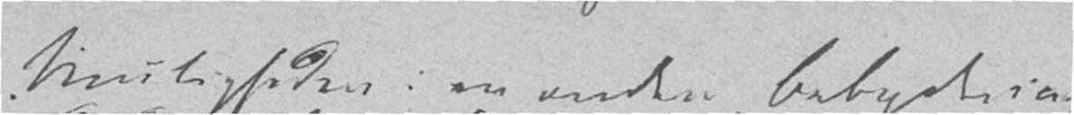Muligheden i en anden Betydning
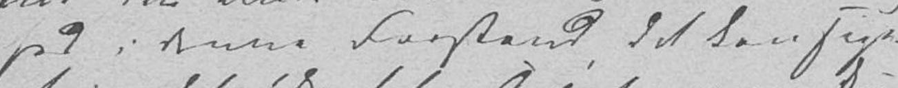hed i denne Forstand, det kan siges
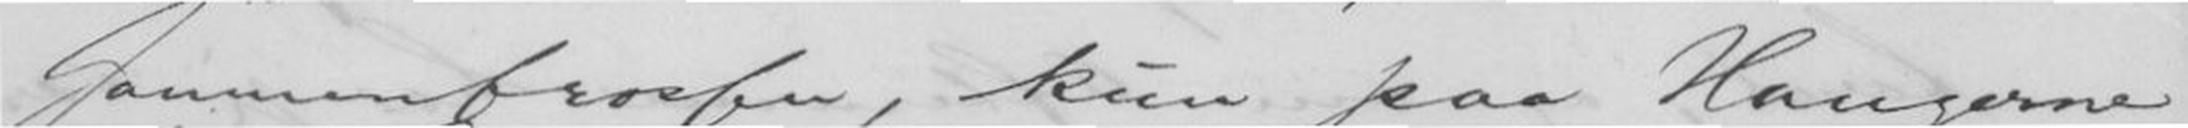sammenfrossen, kun paa Haugerne
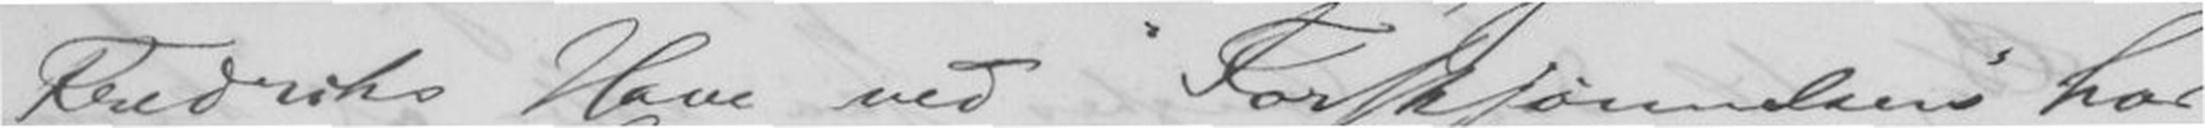Fredriks Have ved "Forskjønnelsen" har
